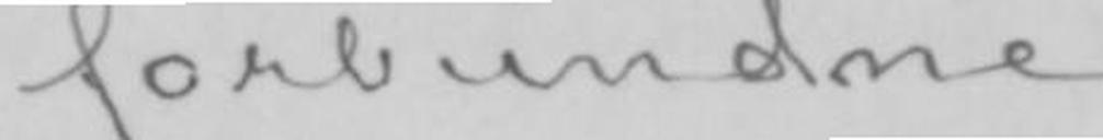forbundne
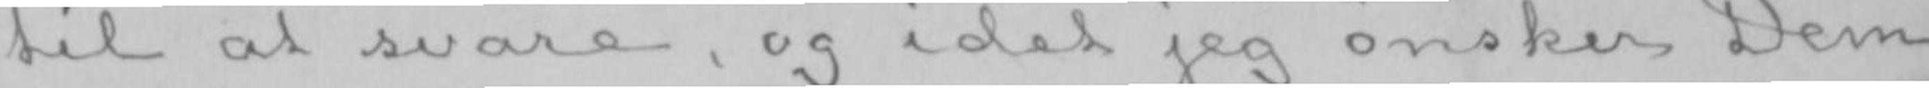til at svare, og idet jeg ønsker Dem
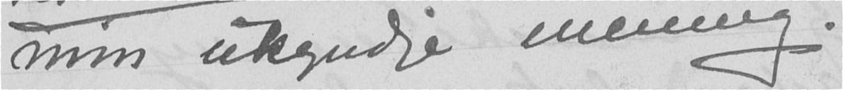min ukyndige mening.
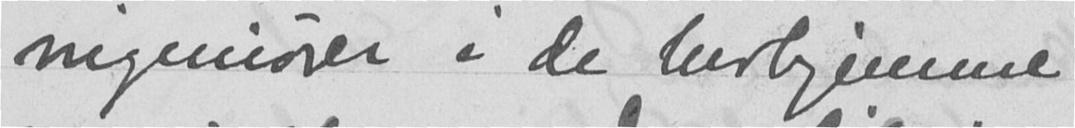ingeniører i de herhjemme
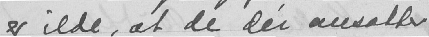er ilde, at de dér ansætter
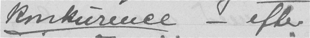konkurence - efter
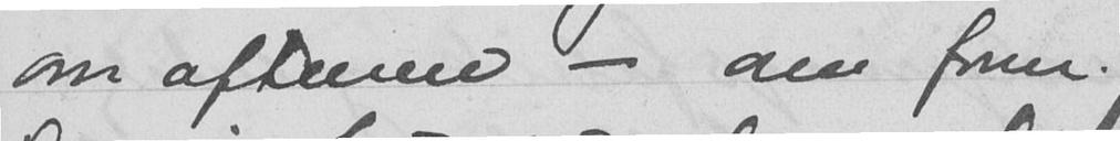om aftenen - om form.
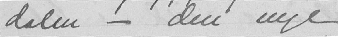dalen - den nye
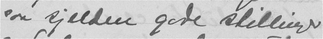saa sjelden gode stillinger
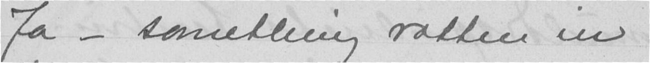Ja - something rotten in
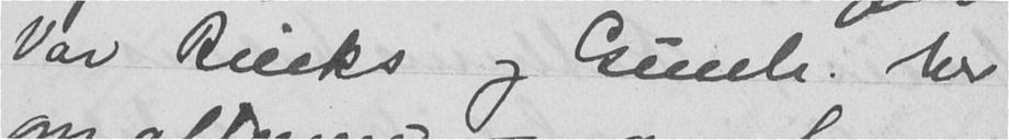Var Riecks og Gunh. her
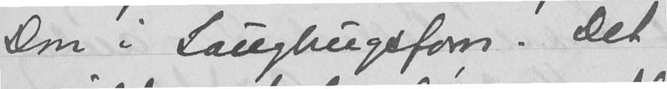Don i Saugbrugsforn. Det
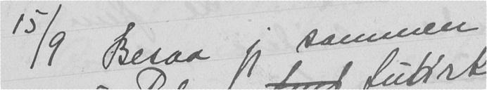15/9 Besaa jeg sammen
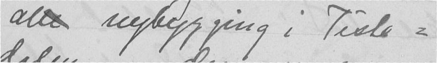alle nybygging i Tiste-
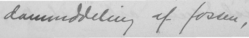damindeling af fossen,
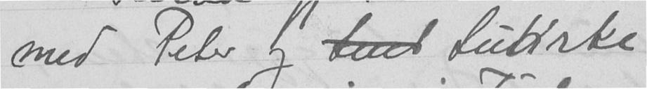med Peter og Surk Suhrke
